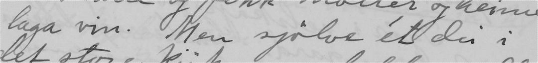laga vin. Men sjölve ét dei i
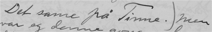Det same på Tinne. Men
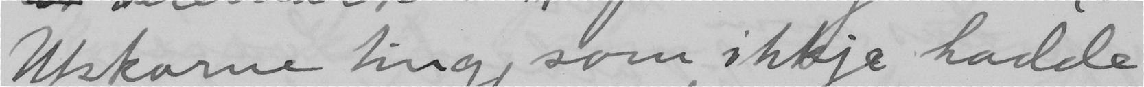Utskarne ting, som ikkje hadde
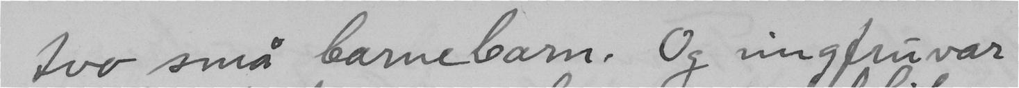tvo små barnebarn. Og ungfruvar
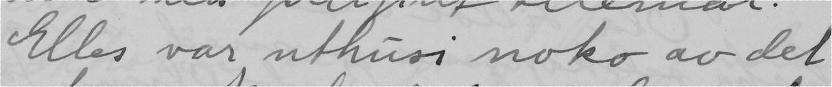Elles var uthusi noko av det
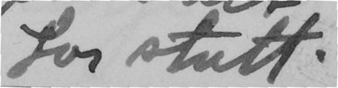for stutt.
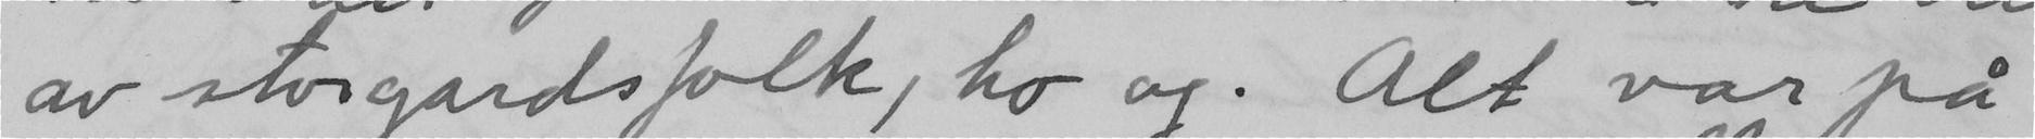av storgardsfolk, ho og. Alt var på
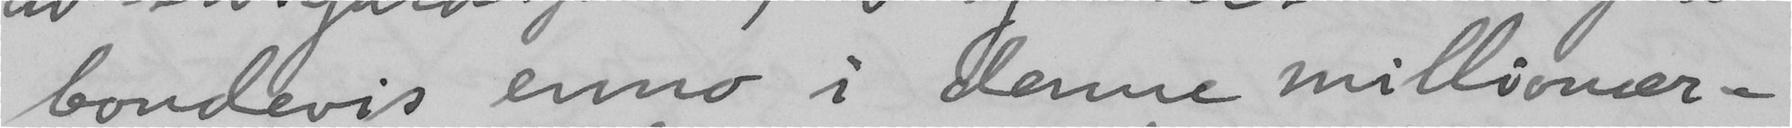bondevis enno i denne millionæ-
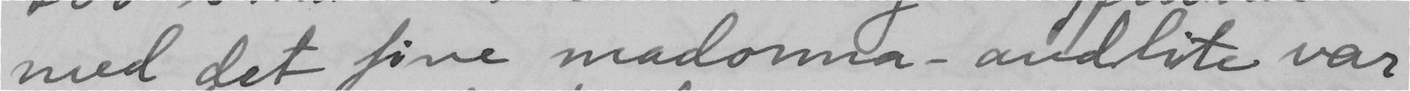med det fine madonna-andlite var
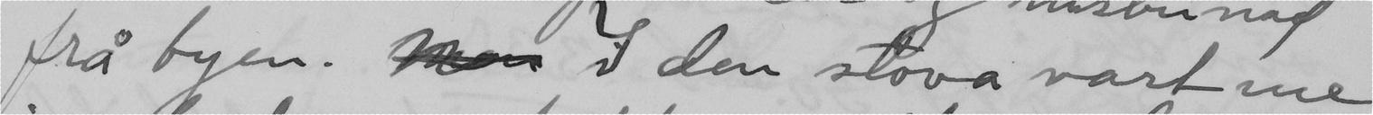frå byen.  I den stova vart me
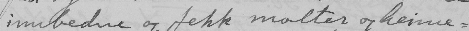innbedne og fekk molter og heime-
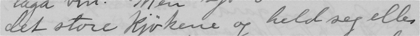det store kjökene og held seg elles
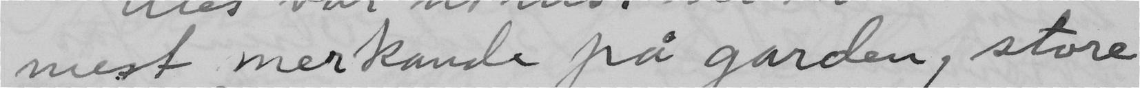mest merkande på garden, store
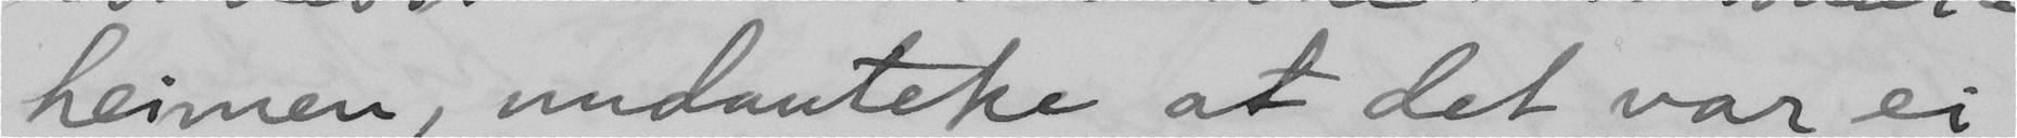heimen, undanteke at det var ei
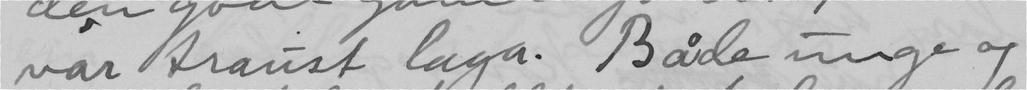var traust laga. Både unge og
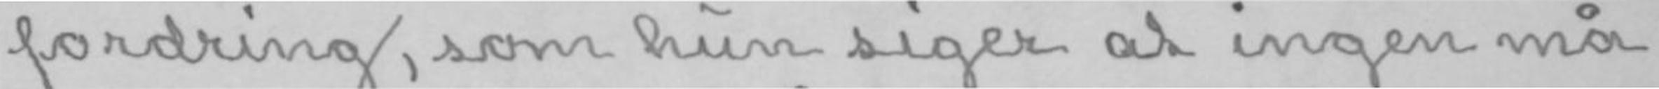fordring, som hun siger at ingen må
